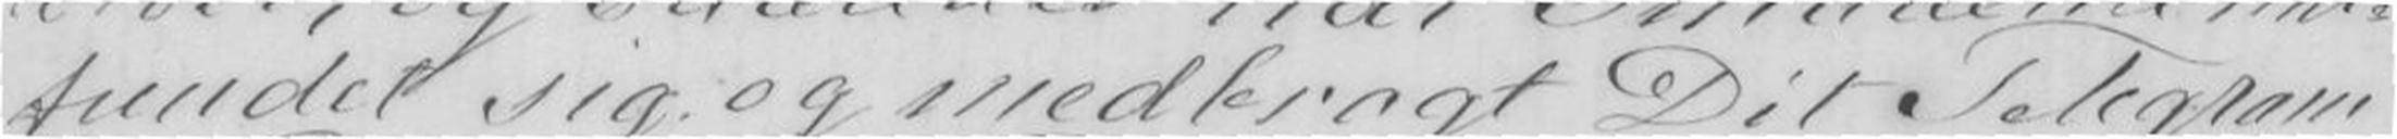fundet sig og medbragt Dit Telegram
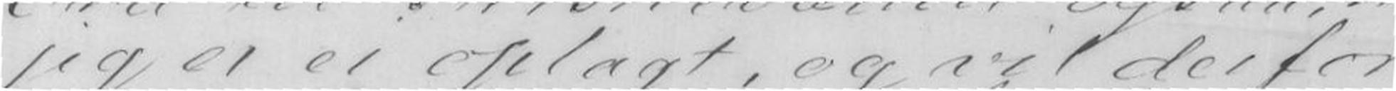jeg er ei oplagt, og vil derfor
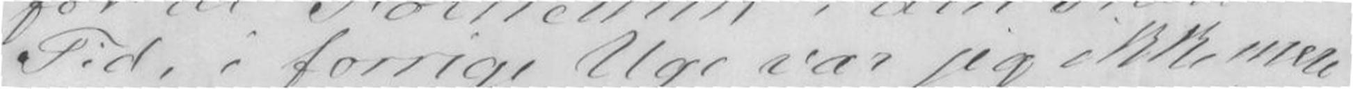Tid, i forrige Uge var vær jeg ikke mere
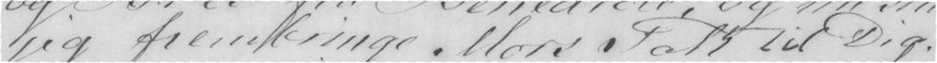jeg frembringe Moer Tak til Dig.
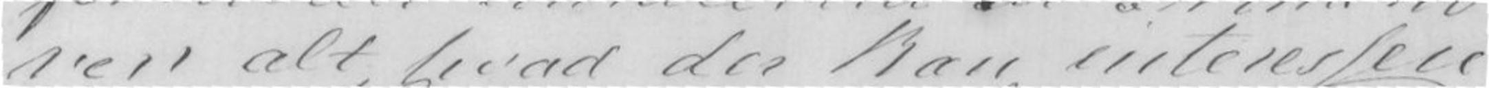rer alt hvad der kan interessere
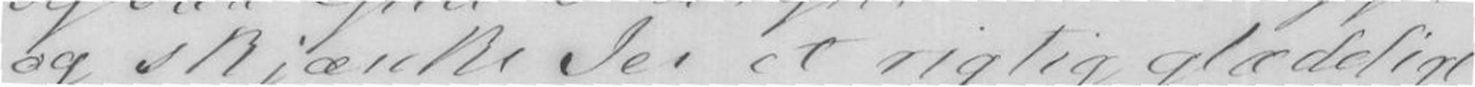og skjænke Jer et rigtig glædeligt
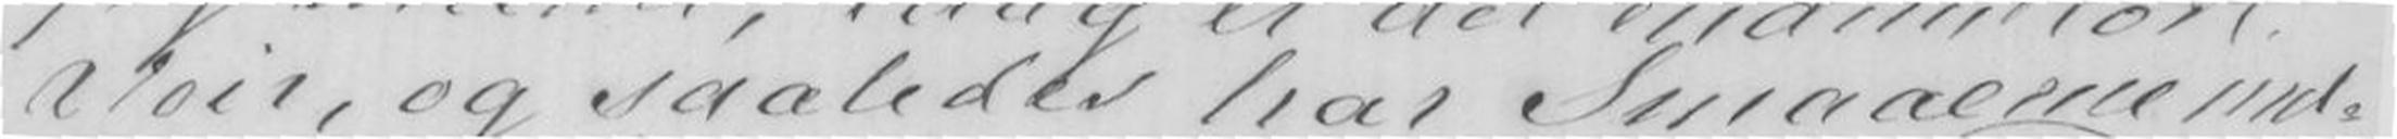Veir, og saaledes har Smaaerne ind-
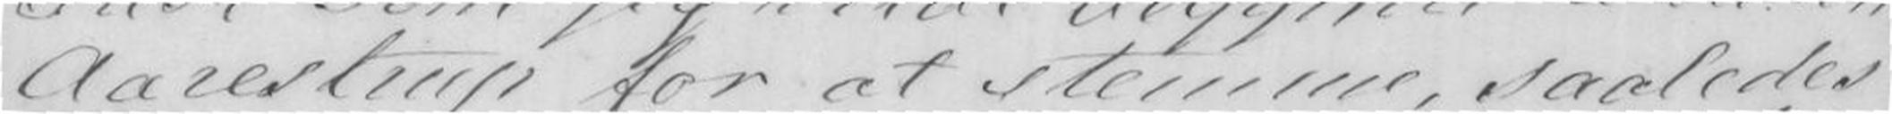Aarestrup for at stemme, saaledes
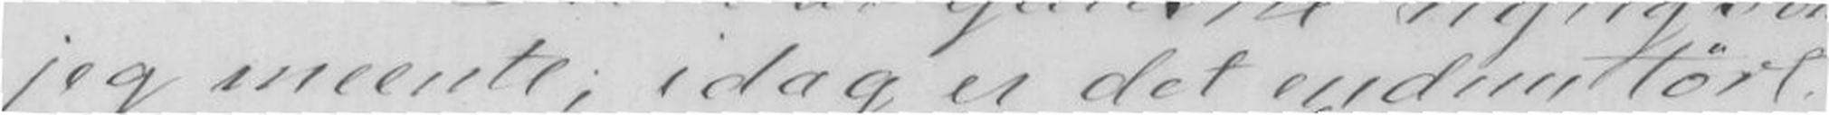jeg mente, idag er det endnu tørt
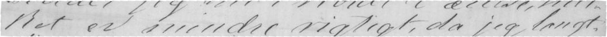ket er mindre rigtig, da jeg langt
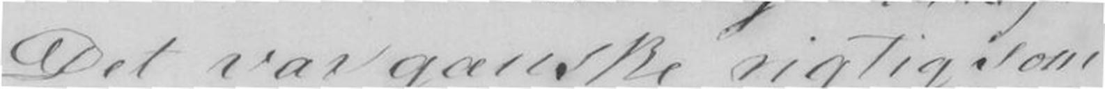Det var ganske rigtig som
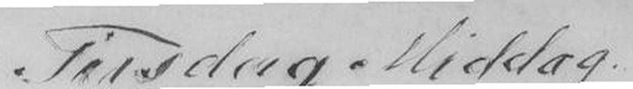Tirsdag Middag
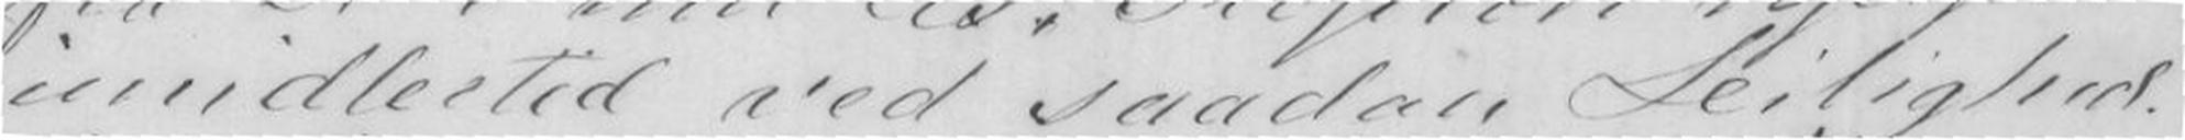imidlertid ved saadan Leilighed.
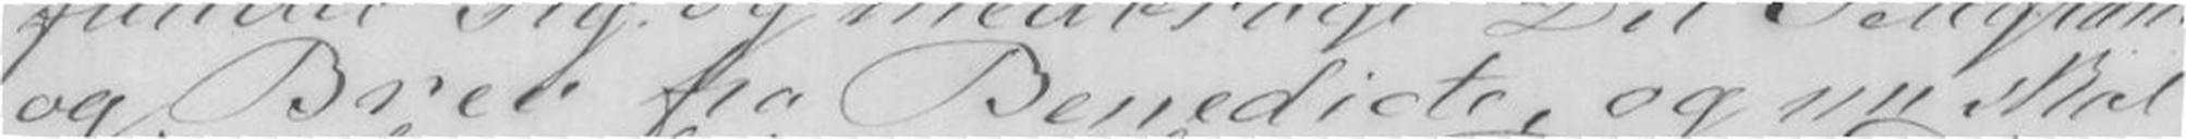og Brev fra Benedicte, og nu skal
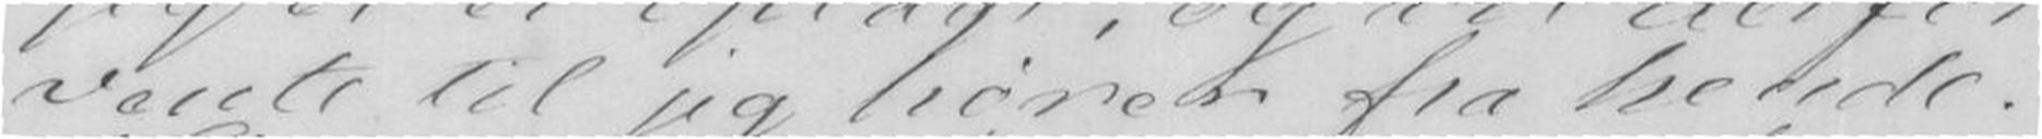vente til jeg hører fra hende.
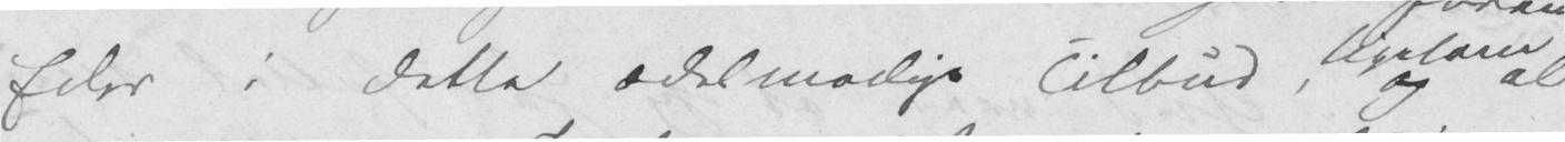Eder i dette ædelmodige Tilbud, ligesom
